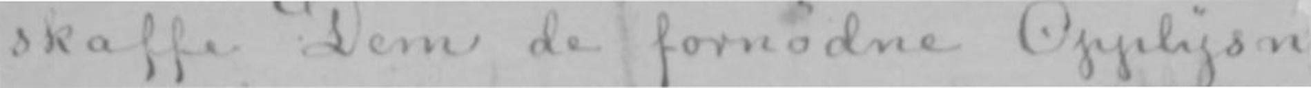skaffe Dem de fornødne Opplysn
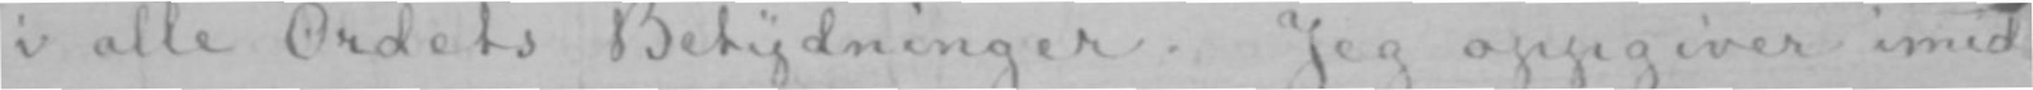i alle Ordets Betydninger. Jeg oppgiver imid-
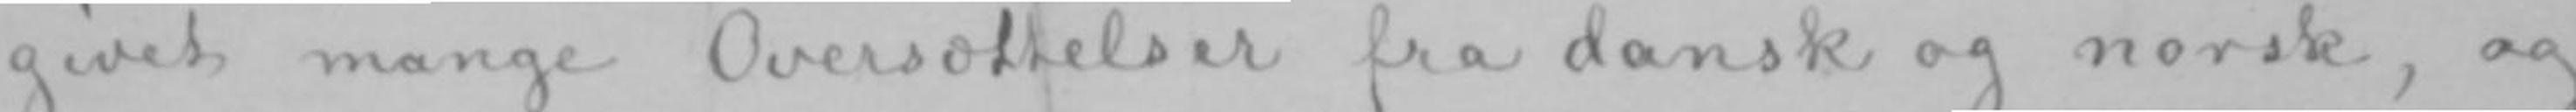givet mange Oversættelser fra dansk og norsk, og
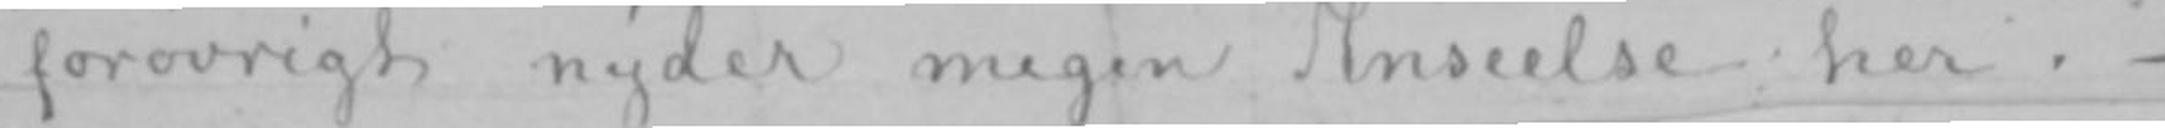forøvrigt nyder megen Anseelse her.-
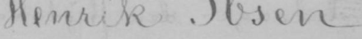Henrik Ibsen.
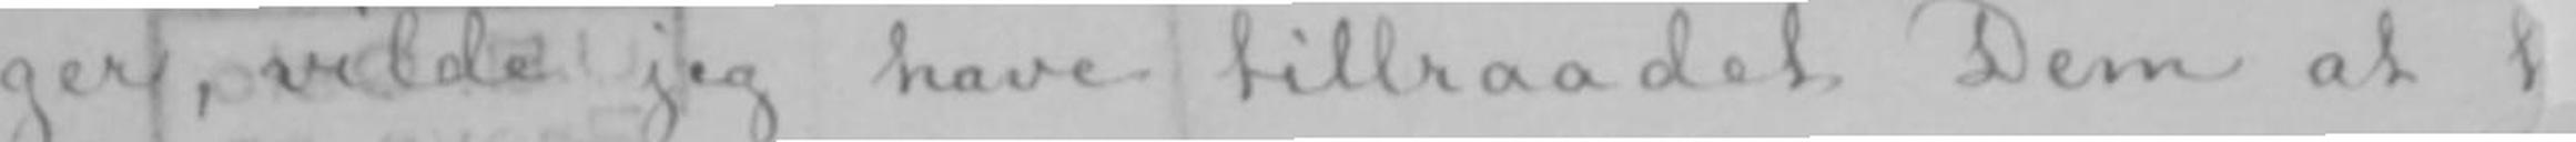ger, vilde jeg have tillraadet Dem at
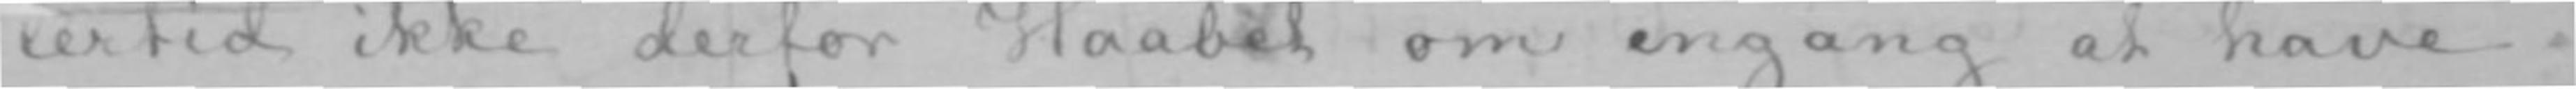lertid ikke derfor Haabet om engang at have
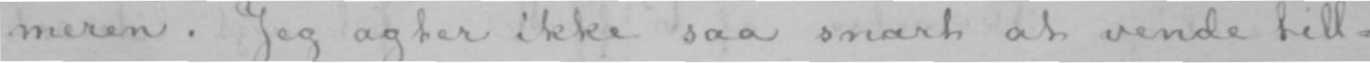meren. Jeg agter ikke saa snart at vende till-
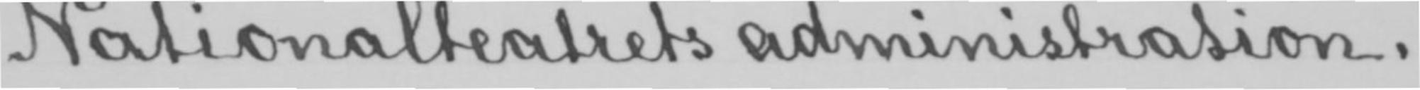Nationalteatrets administration.
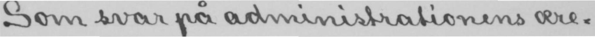Som svar på administrationens ære-
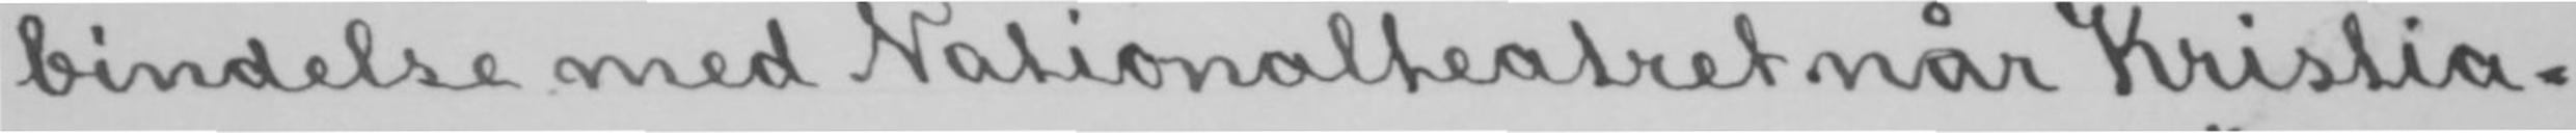bindelse med Nationalteatret når Kristia-
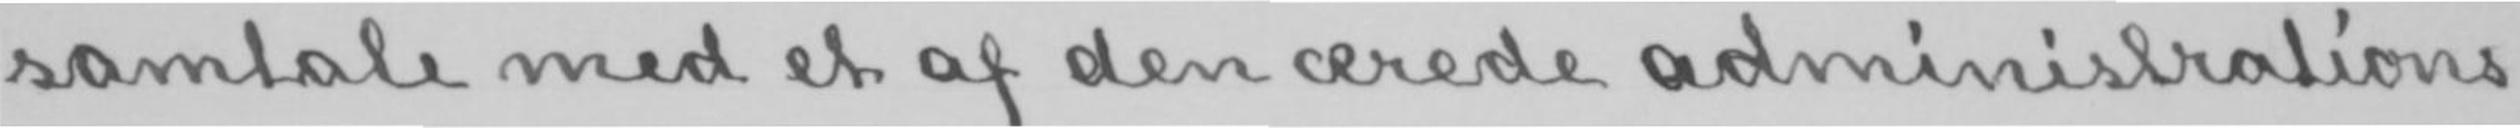samtale med et af den ærede administrations
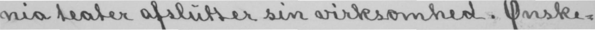nia teater afslutter sin virksomhed. Ønske-
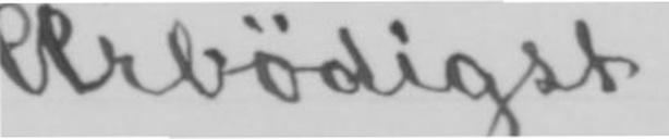Ærbødigst
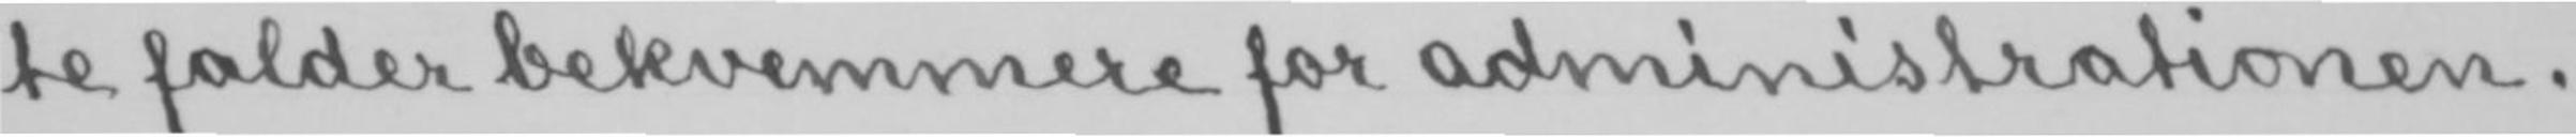te falder bekvemmere for administrationen.
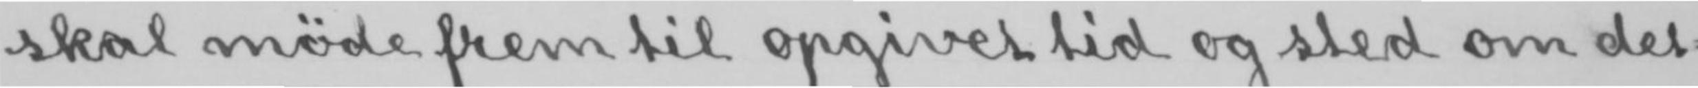skal møde frem til opgivet tid og sted om det-
